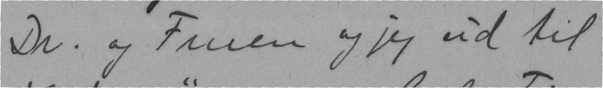Dr. og Fruen og jeg ud til
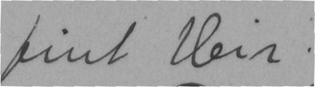fint Veir.
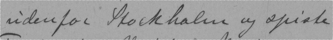udenfor Stockholm og spiste
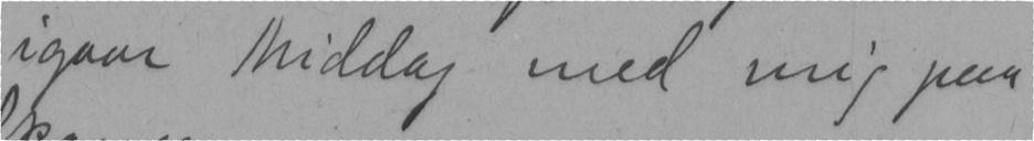igaar Middag med mig paa
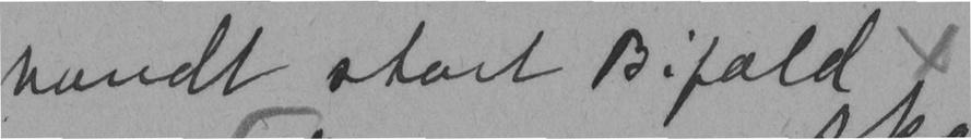vandt stort Bifald x
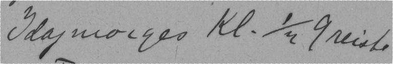Idagmorges Kl. 1/2 9 reiste
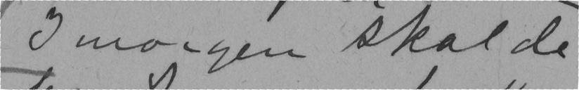I morgen skal de
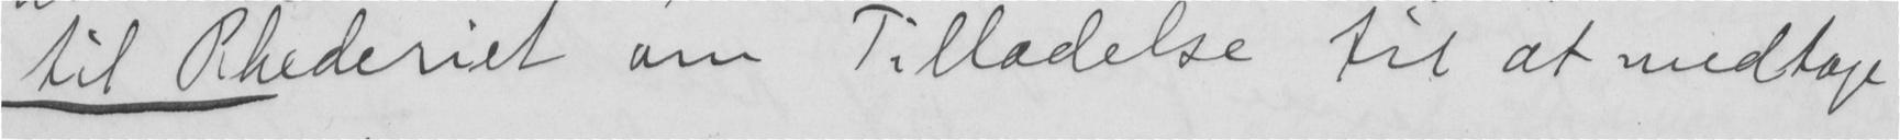til Rhederiet om Tilladelse til at medtage
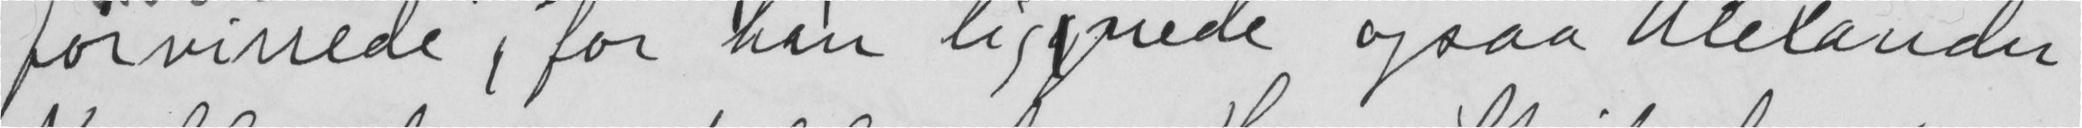forvirrede, for han lignede ogsaa Alexander
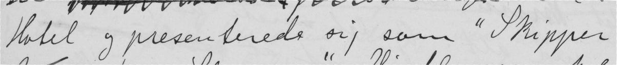Hotel og presenterede sig som "Skipper
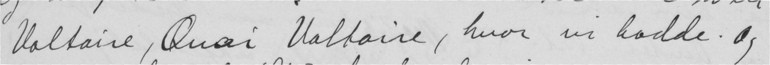Voltaire, Quai Valtoire, hvor vi bodde. Og
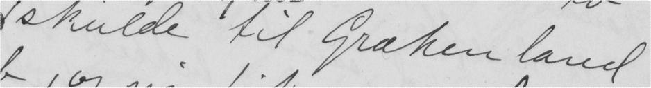skulde til Grækenland
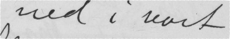ned i vort
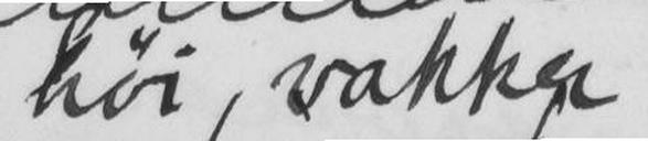høi, vakker
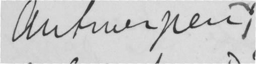Antwerpen;
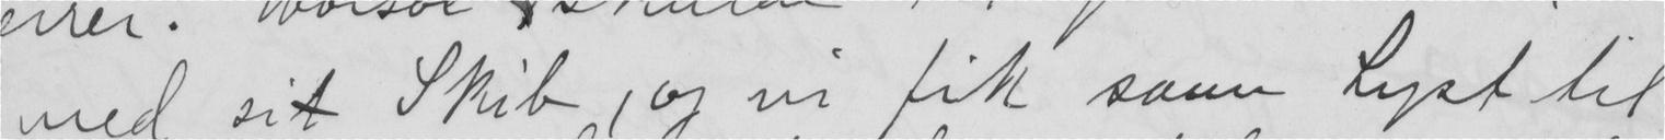med sit Skib, og vi fik saan Lyst til
